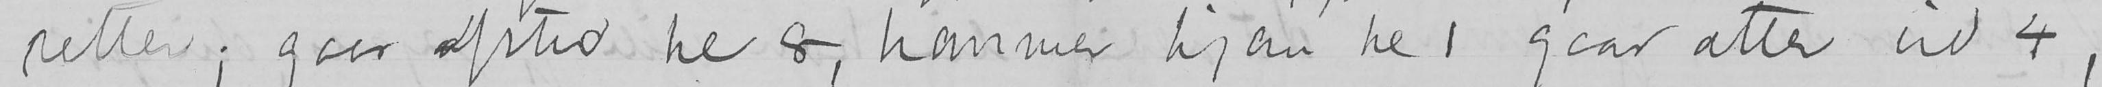retter; gaar afsted kl 8, kommer hjem kl 1 gaar atter ud 4,
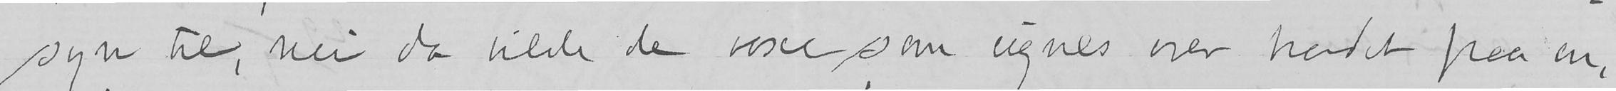syn til, nei da vilde de voxe som ugres over hodet paa en,
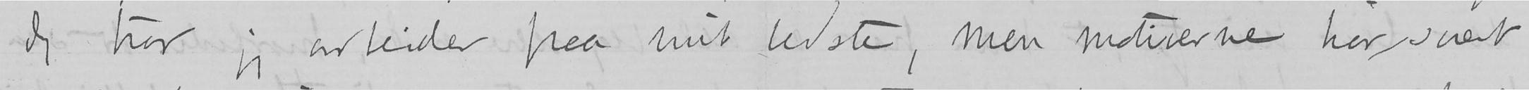Jeg tror jeg arbeider paa mit bedste, men motiverne har svært
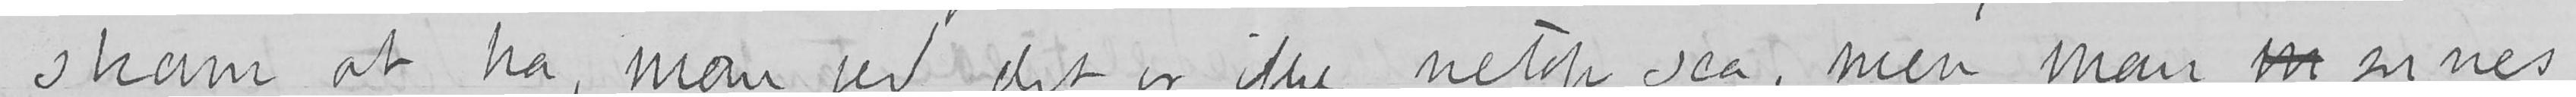skam at ha, man ved det er ikke netop saa, men man m synes
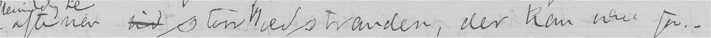aftenen sid staar jeg ved stranden, der kan være for-
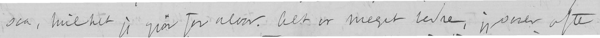saa, hvilket jeg gjør for alvor. Alt er meget bedre, jeg sover ofte
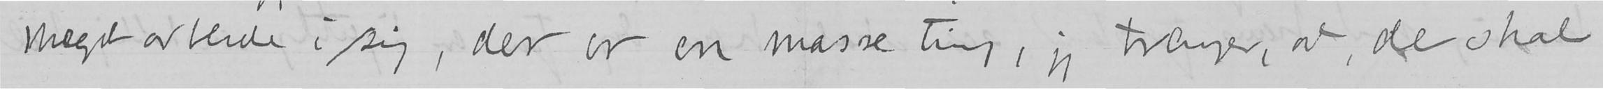meget arbeide i sig, der er en masse ting, jeg trænger, at, de skal
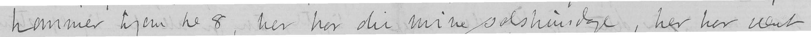kommer hjem kl 8, her har du mine solskinsdage, her har været
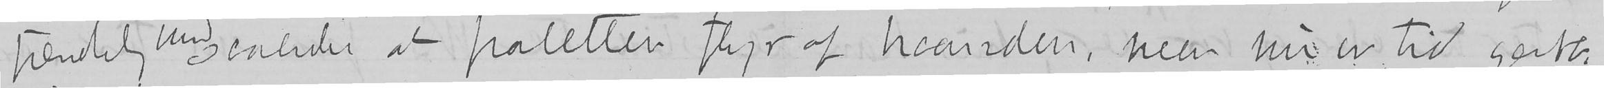færdelig vind saaledes at paletten flyr af haanden, men nu er tid godt.
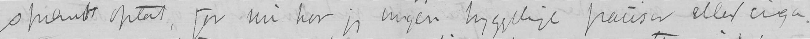spændt optat, for nu har jeg ingen hyggelige pauser eller ciga-
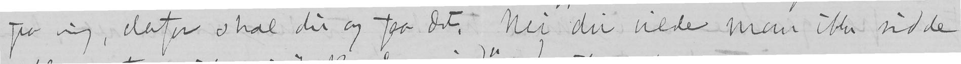fra mig, derfor skal du og faa det. Nei du vilde man ikke sidde
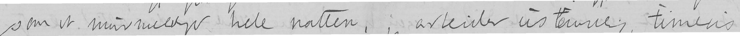som et murmeldyr hele natten, jeg arbeider ustanselig, timevis
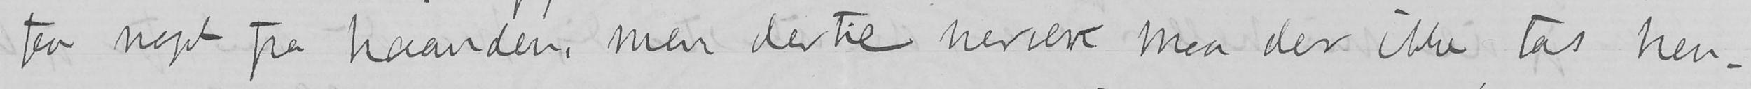faa noget fra haanden, men dertil nerven maa der ikke tas hen-
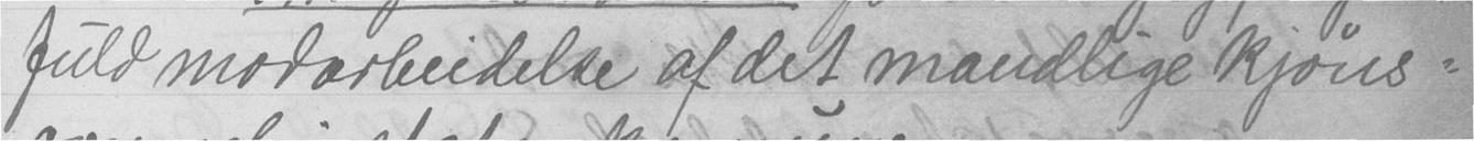fuld modarbeidelse af det mandlige kjøns-
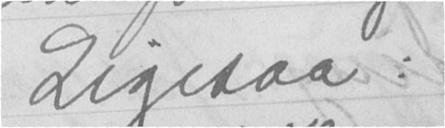Ligesaa:
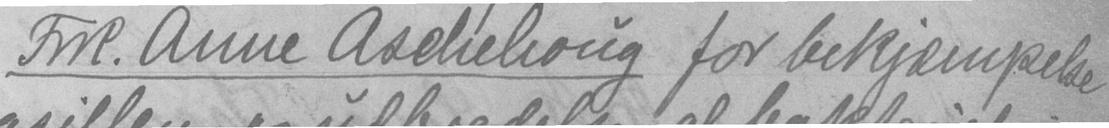Frk. Anne Aschehoug for bekjæmpelse
